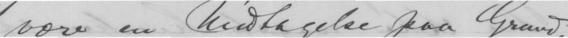være en Undtagelse paa Grund
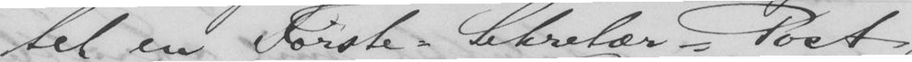tet en Første=Sekretær=Post
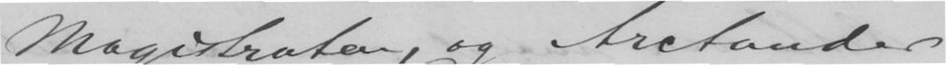Magistraten og Arctander
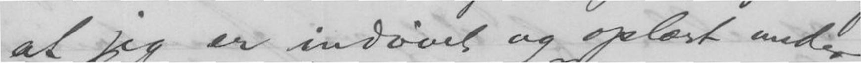af at jeg er indøvet og oplært under
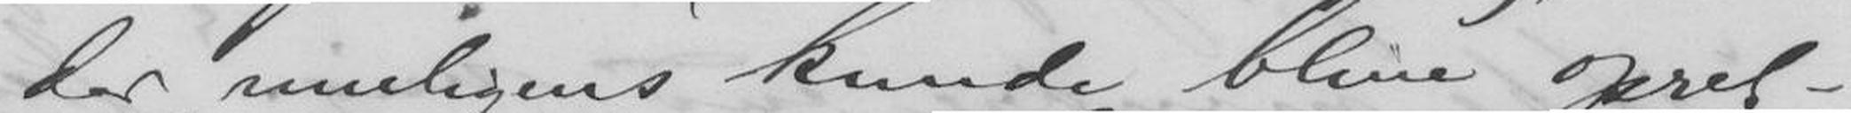der muligens kunde blive opret-
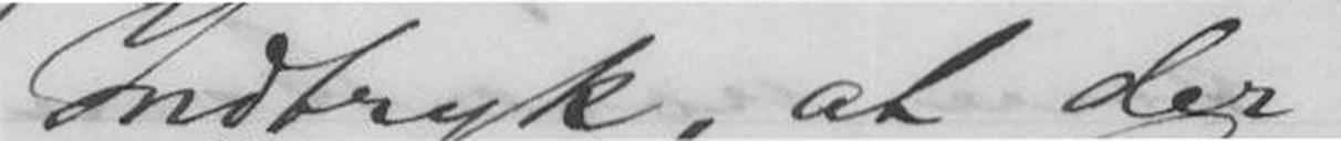Indtryk, at der
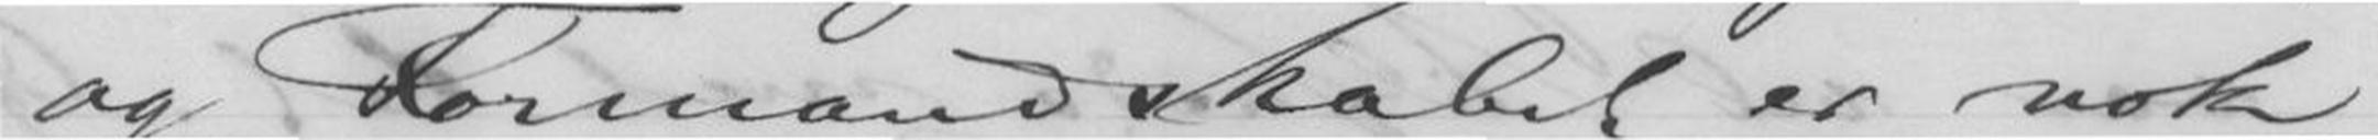og Formandskabet er nok
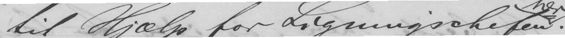til Hjælp for Ligningschefen her.
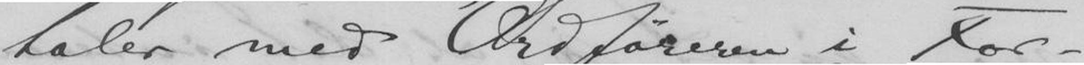taler med Ordføren i For-
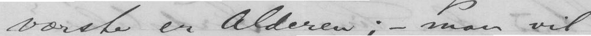værste er Alderen; - man vil
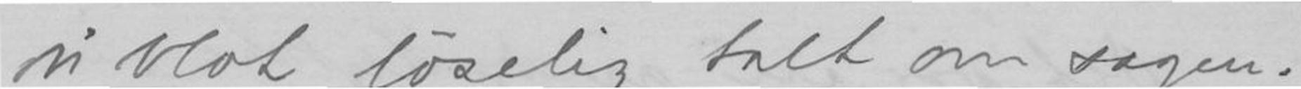vi blot løselig talt om sagen.
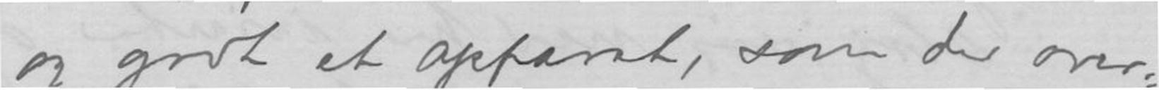og godt et apparat, som der over-
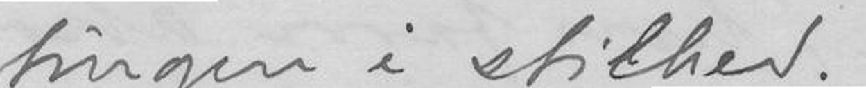tingen i stilled.
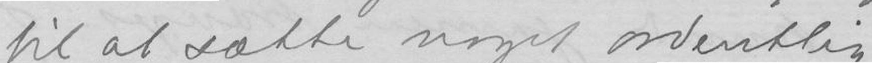til at sætte nogel ordentlig
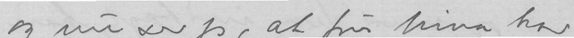og nu ser jeg, at fru Nina har
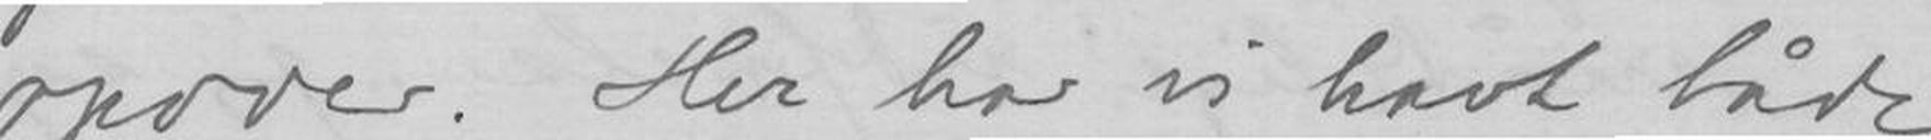opover. her har vi havt både
